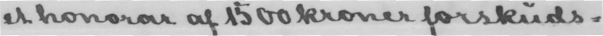et honorar af 1500 kroner forskuds-
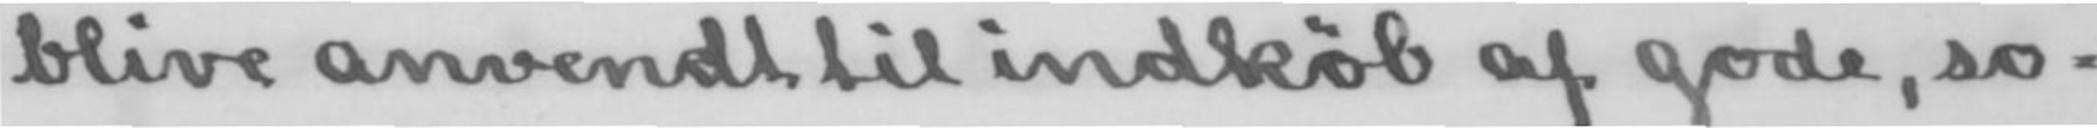blive anvendt til indkøb af gode, so-
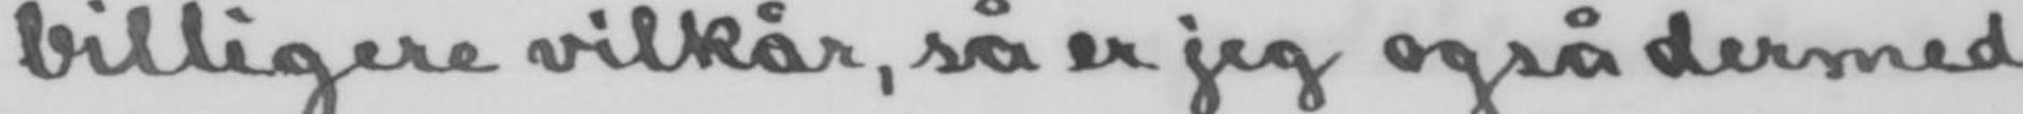billigere vilkår, så er jeg også dermed
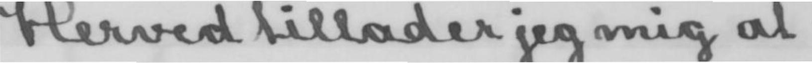Herved tillader jeg mig at
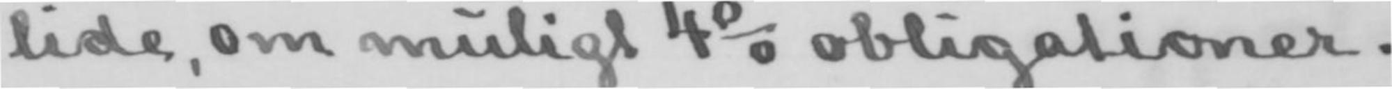lide, om muligt 4% obligationer.
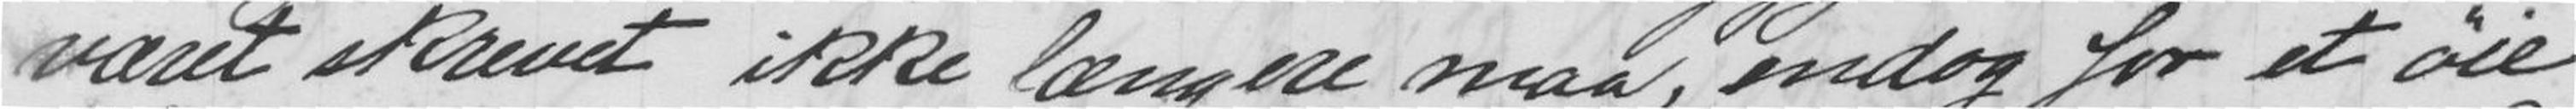været skrevet ikke længere maa, endog for et øie
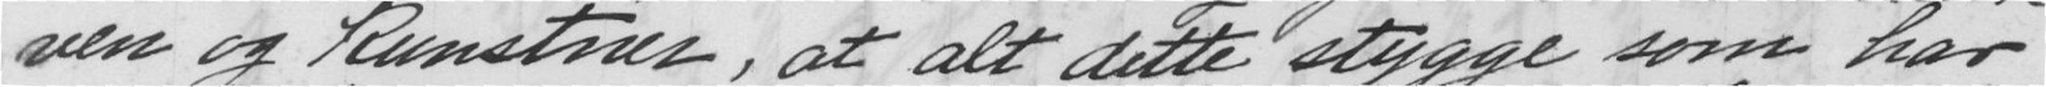ven og kunstner, at alt dette stygge som har
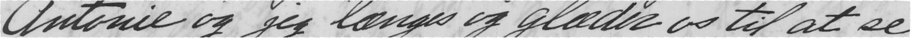Antonie og jeg længes og glæder os til at se
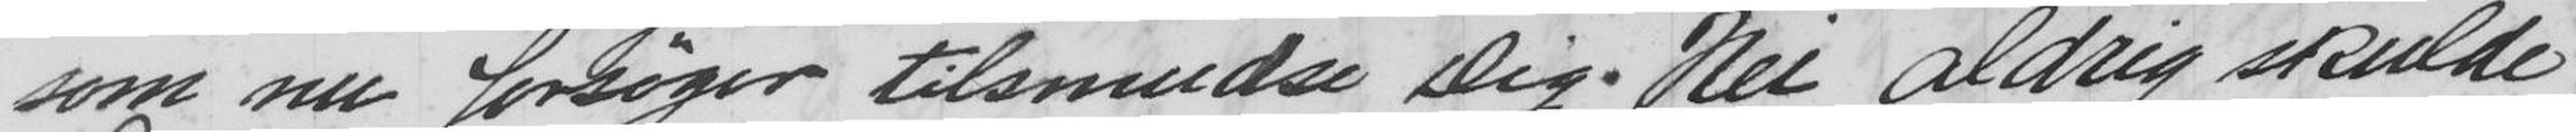som nu forsøger tilsmudse Dig. Nei aldrig skulde
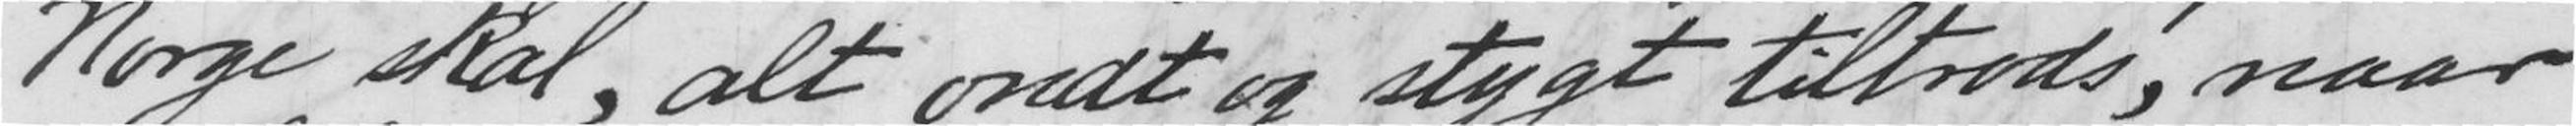Norge skal, alt ondt og stygt tiltrods, naar
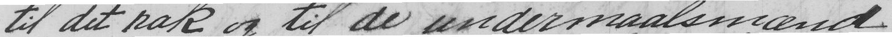til det pak og til de undermaalsmænd
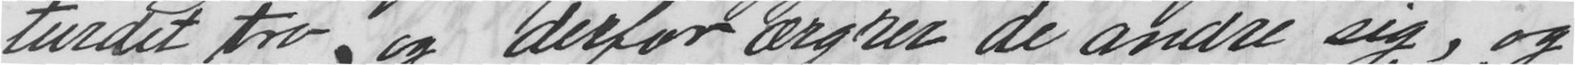turdet tro, og derfor ærgrer de andre sig, og
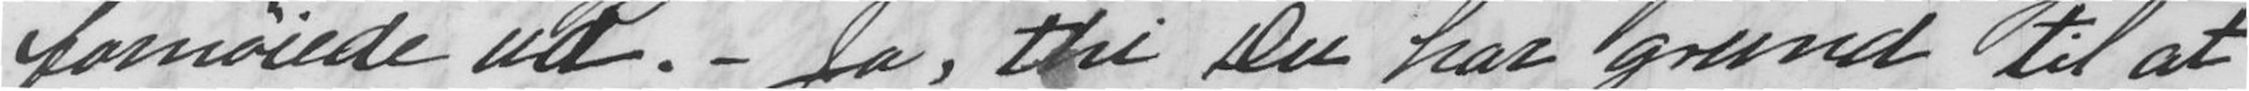fornøiede ud. - Ja, thi Du har grund til at
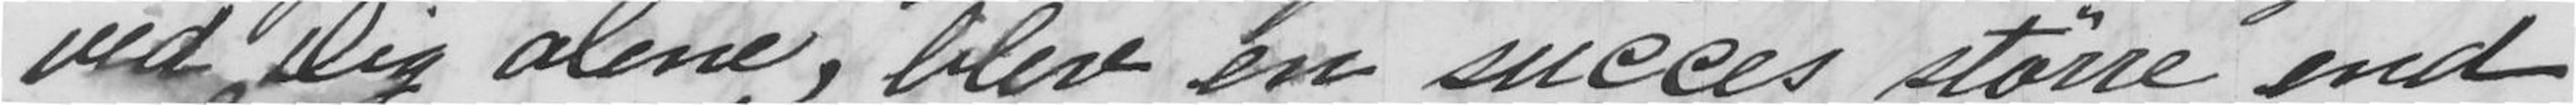ved Dig alene, blev en succes større end
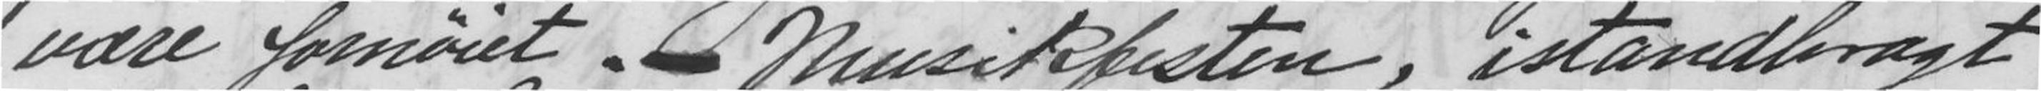være fornøiet. - Musikfesten, istandbragt
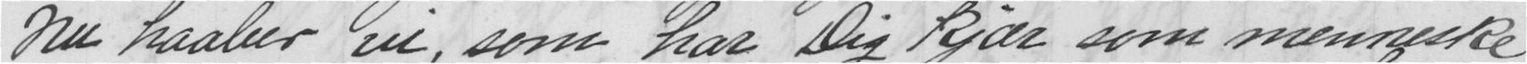Nu haaber vi, som har Dig kjær som menneske
